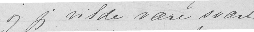og jeg vilde være svært
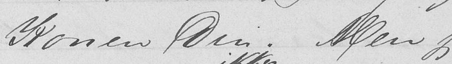Konen Din. Men jeg
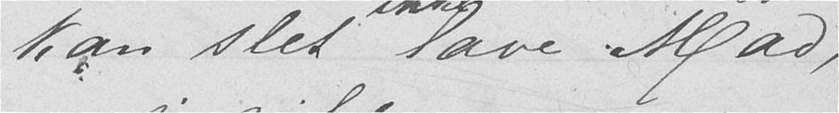kan slet lave Mad,
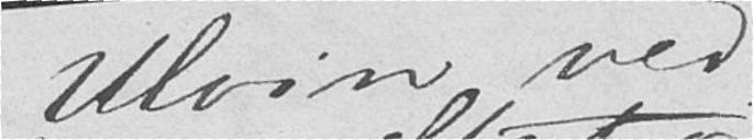Ulvin ved
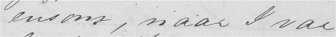ensom, naar I var
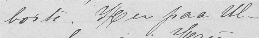borte. Her paa Ul-
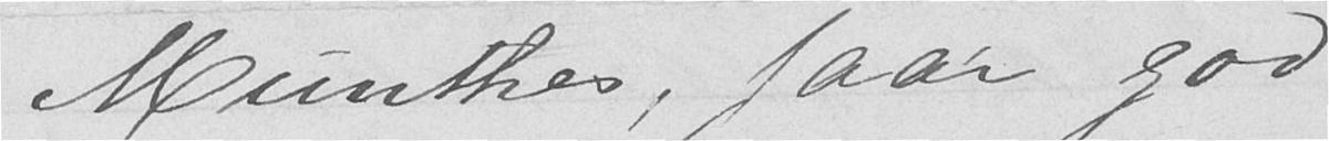Munthes, faar god
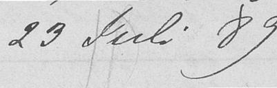23 Juli 89
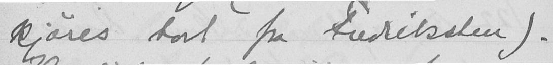kjøres bort fra Fredriksten).
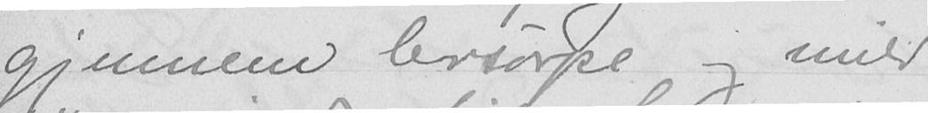gjennem lersørpe og mild
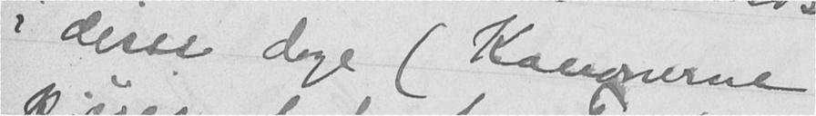i disse dage (Kanonerne
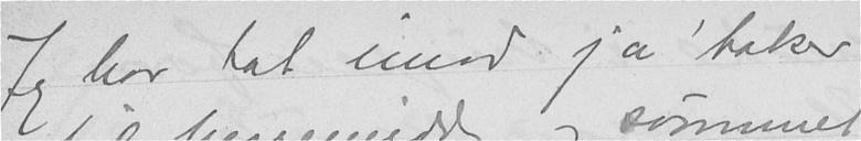Jeg har tat imod ja 'kaker
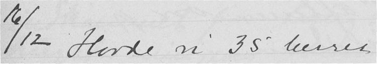16/12 Havde vi 35 herrer
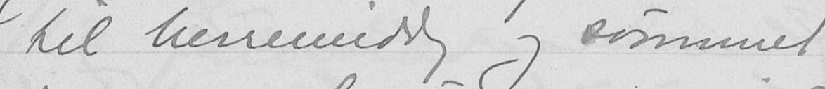til herremiddag og svømmet
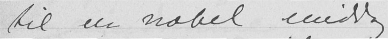til en nobel middag
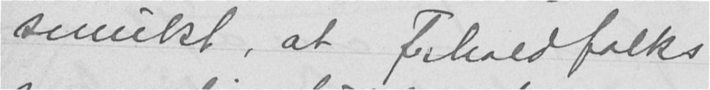smukt, at Frhaldfolks
